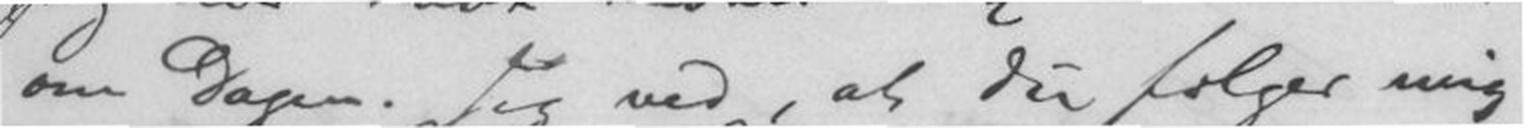om Dagen. Jeg ved, at du følger mig
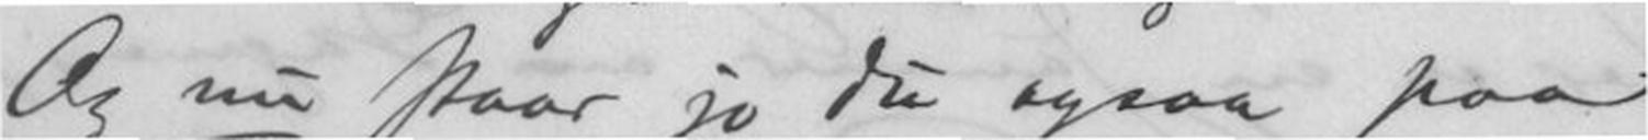Og nu staar jo du ogsaa paa
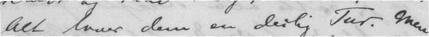Alt lover dem en deilig Tur. Men
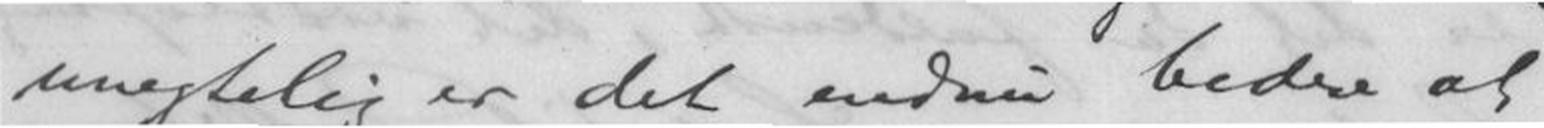unegtelig er det endnu bedre at
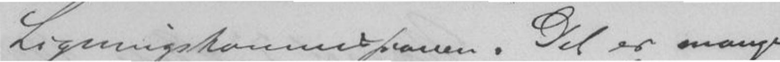Ligningskommissionen. Det er mange
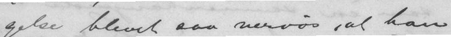gelse blevet saa nervøs, at han
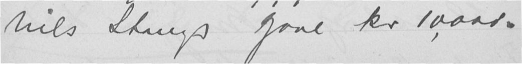Nils Stangs gave kr 10,000.
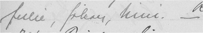Julie, Johan, Nini. -
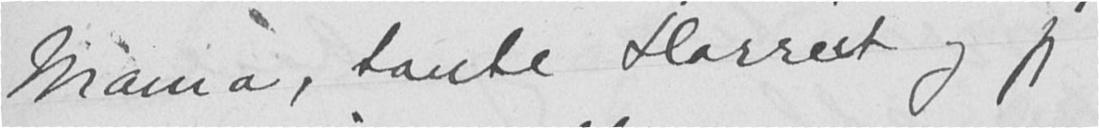Mama, tante Harriet og jeg
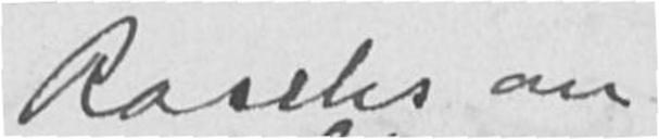Raschs om
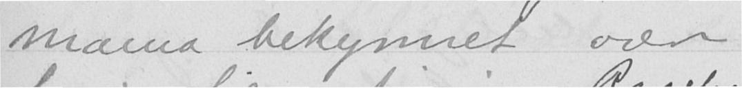mama bekymret over
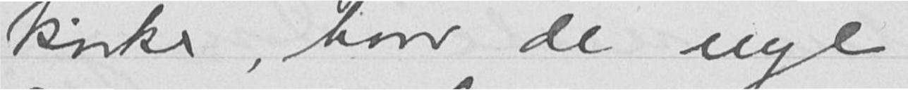kirke, hvor de nye
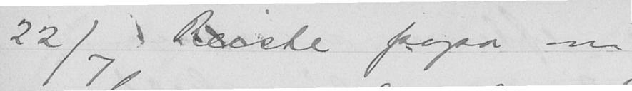22/7 Reiste papa om
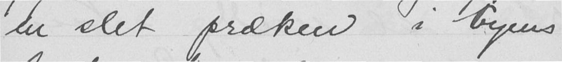en slet præken i byens
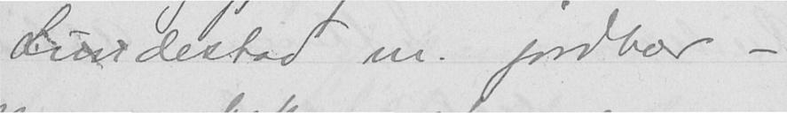Lundestad m. jordbær -
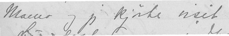Mama og jeg kjørte visit
